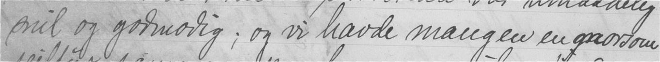mil og godmodig; og vi havde mangen en morsom
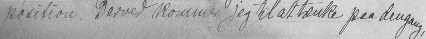position. Derved kommer jeg til at tænke paa dengang,
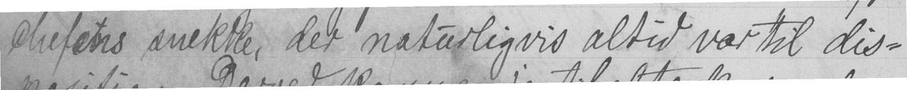chefens snekke, der naturligvis altid var til dis-
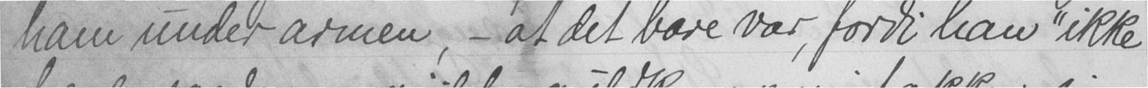ham under armen, - at det bare var, fordi han "ikke
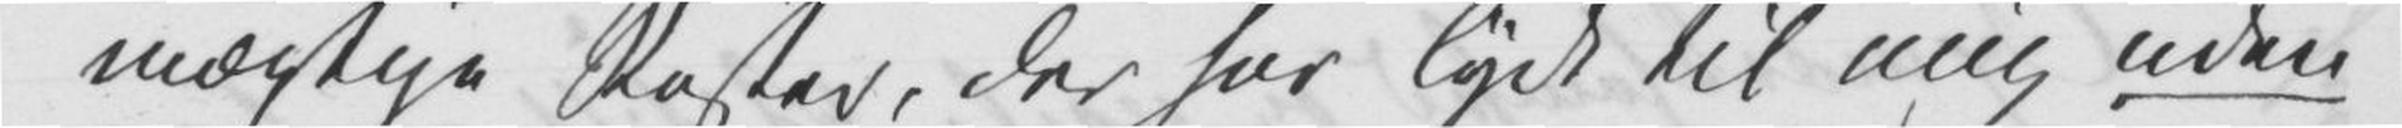mægtige Røster, der har lydt til mig uden¬
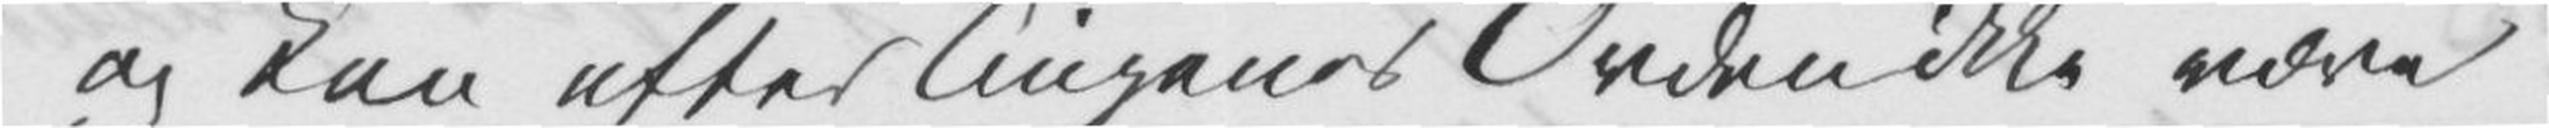og kan efter Tingenes Orden ikke være
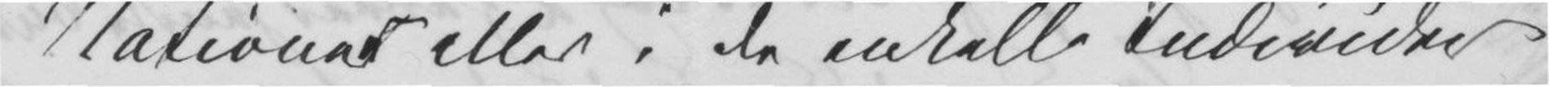Nationer eller i de enkelte Individer
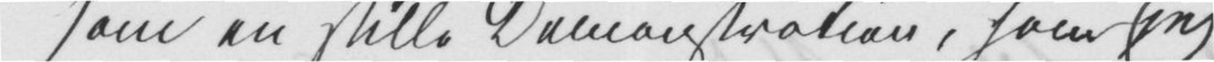som en stille Demonstration, som jeg
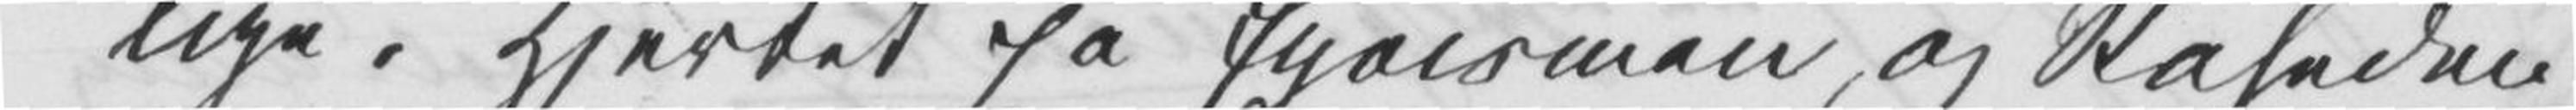lige i Hjertet på Egoismen og Råheden
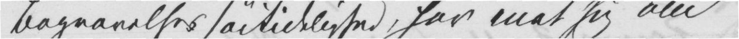Begravelseshøitidelighed, har enet sig om
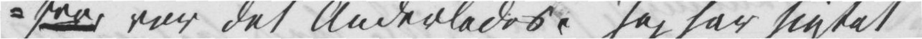fra var det Anderledes. Jeg har sigtet
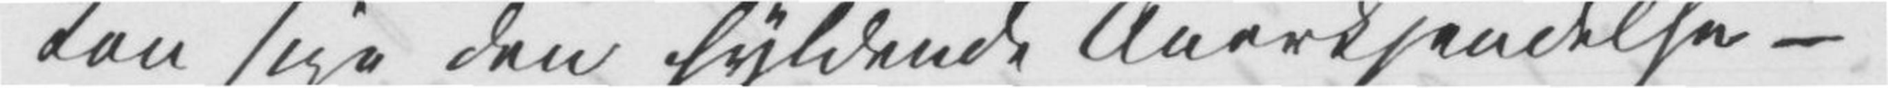kan sige den hyldende Anderkjendelse –
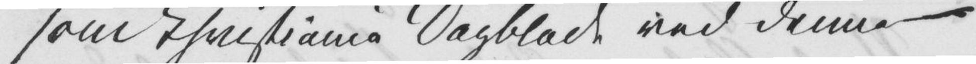som Christiania Dagblad ved denne
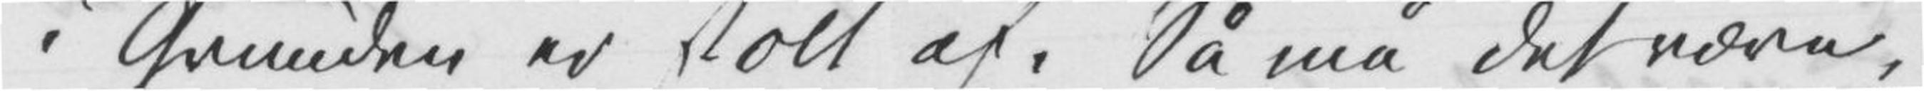i Grunden er stolt af. Så må det være,
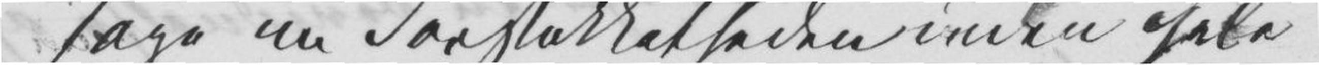søge nu Forstokketheden inden hele
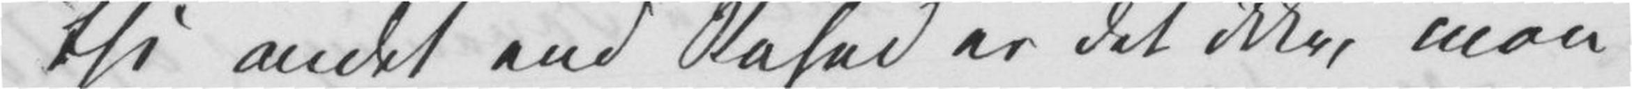thi andet end Råhed er det ikke, man
